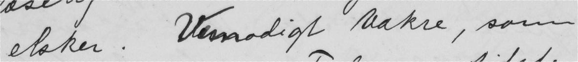elsker. Vemodigt Vakre, som
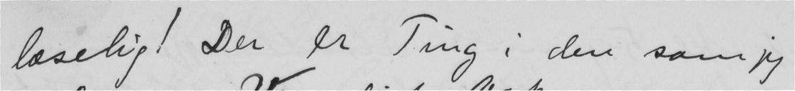læselig! Der er Ting i den som jeg
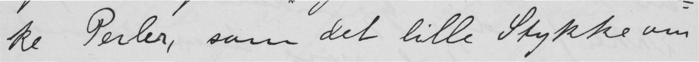ke Perler, som det lille Stykke om
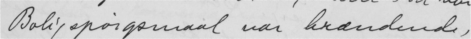Bolig spørgsmaal var brændende,
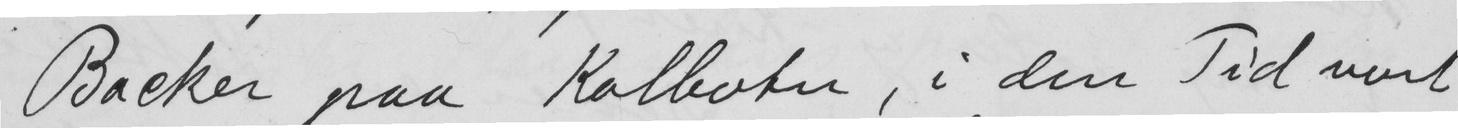Backer paa Kolbotn, i den Tid vort
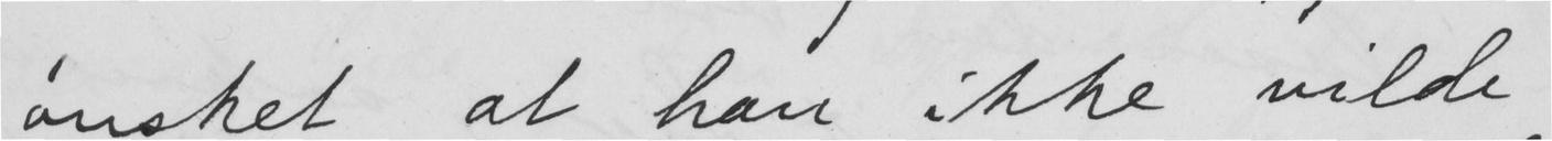ønsket at han ikke vilde
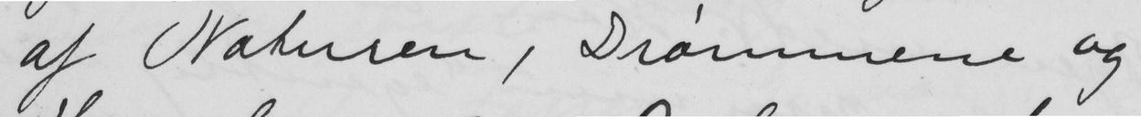af Naturen, Drømmene og
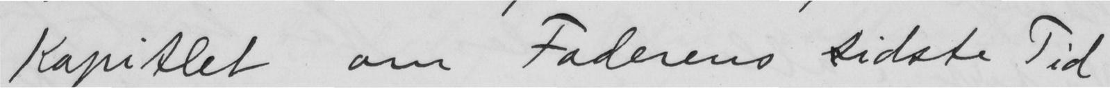Kapitlet om Faderens sidste Tid
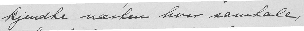kjendte næsten hver samtale,
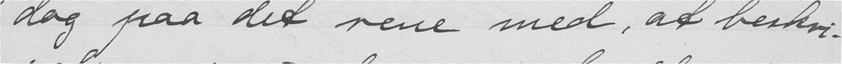dog paa det rene med, at beskri-
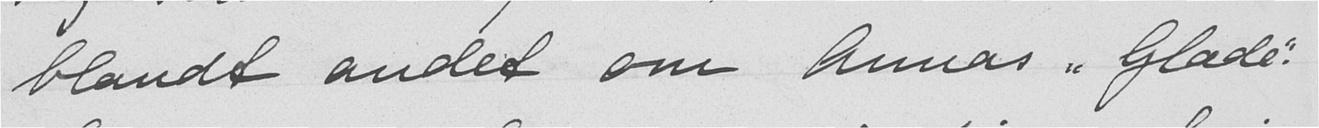blandt andet om Annas "Glæde".
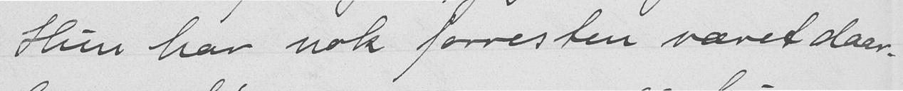Hun har nok forresten været daar-
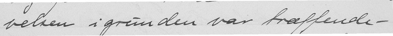velsen igrunden var træffende -
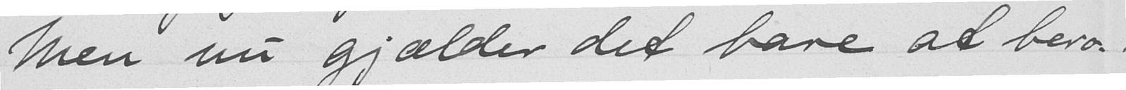Men nu gjælder det bare at bero-
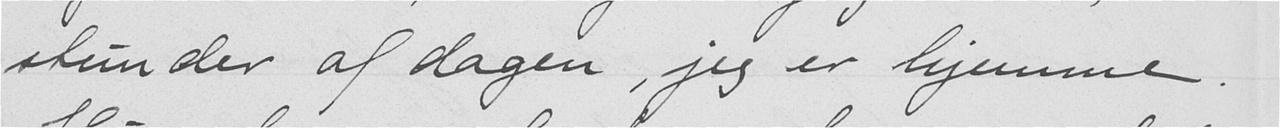stunder af dagen, jeg er hjemme.
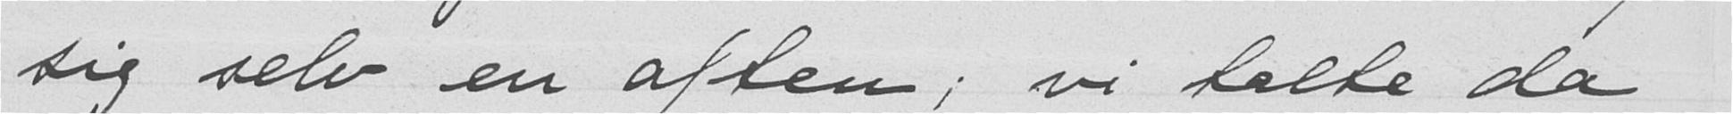sig selv en aften; vi talte da
